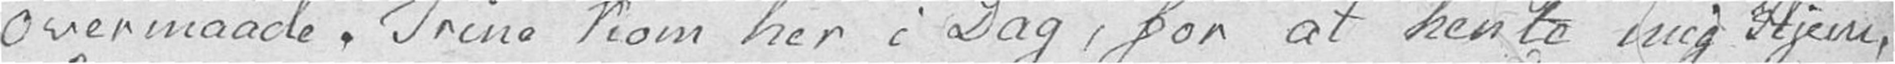overmaade. Trine kom her i Dag, for at hente mig Hjem,
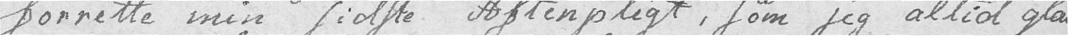forrette min sidste Aftenpligt, som jeg altid glad
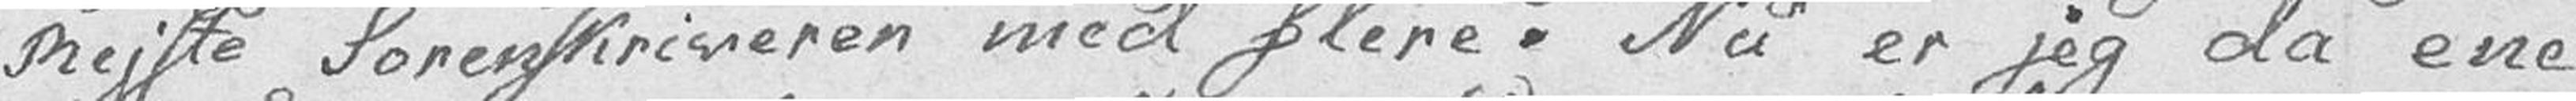Rejste Sorenskriveren med flere. Nu er jeg da ene
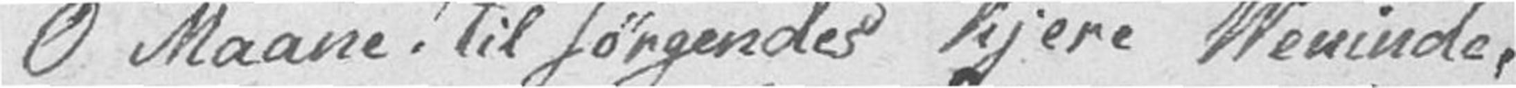O Maane! til sørgendes kjere Veninde,
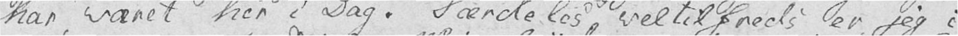har været her i Dag. Særdeles veltilfreds er jeg i
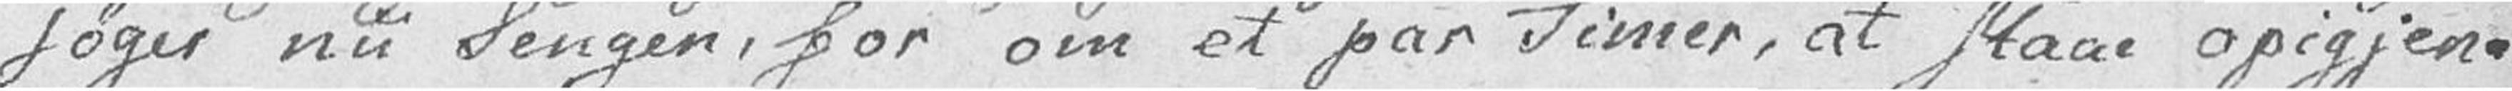søger nu Sengen, for om et par Timer, at staae op igjen,
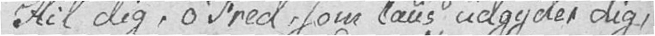Hil dig, o Fred, som taus udgyder dig,
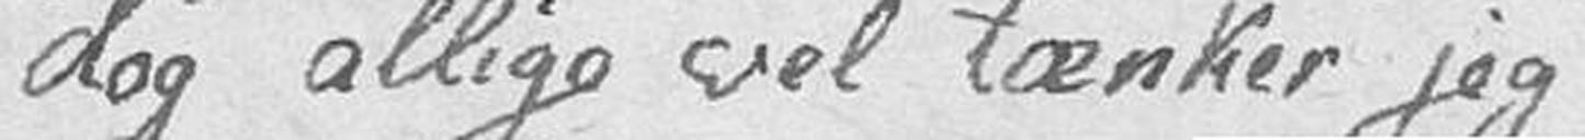dog allige vel tænker jeg
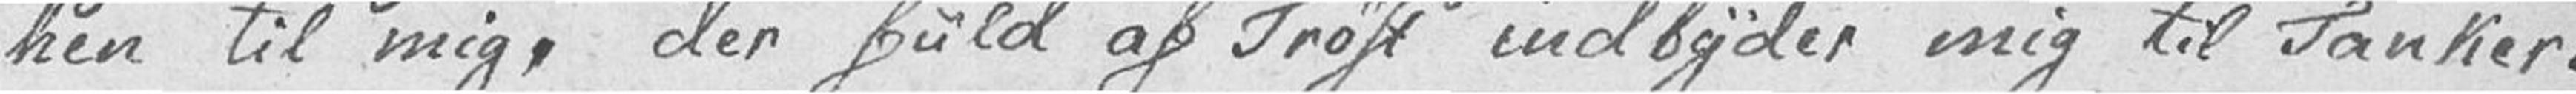hen til mig, der fuld af Trøst indbyder mig til Tanker.
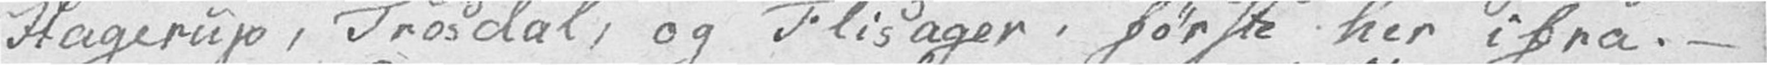Hagerup, Trosdal og Flisager, første her ifra. –
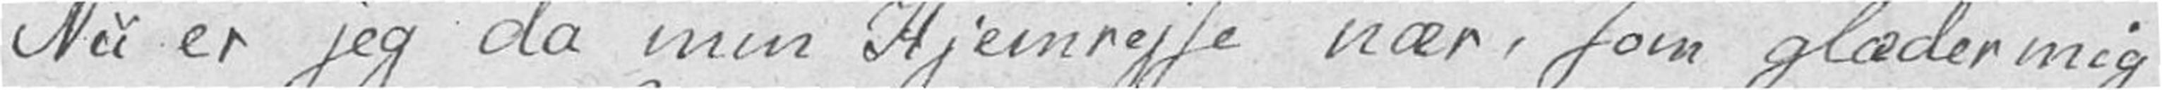Nu er jeg da min Hjemrejse nær, som glæder mig
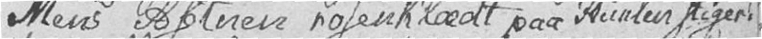Mens Aftnen rosenklædt paa Himlen stiger!
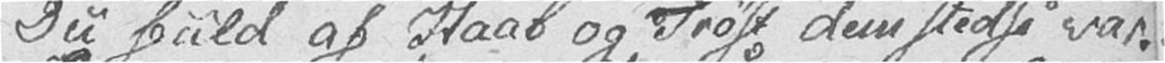Du fuld af Haab og Trøst dem stedse var.
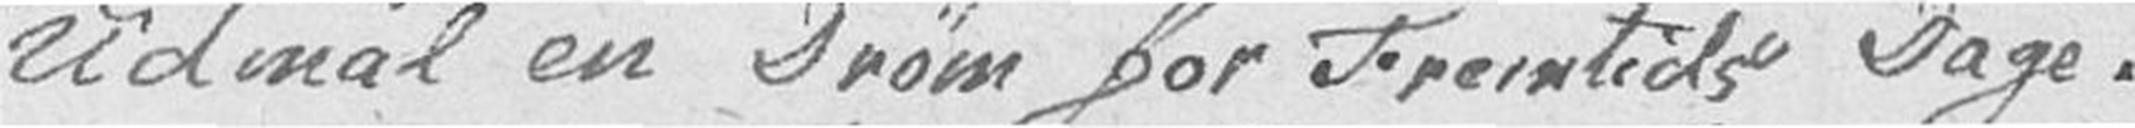Udmal en Drøm for Fremtids Dage!
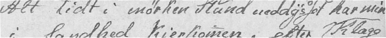Alt tidt i mørken Stund neddysset har min Klage
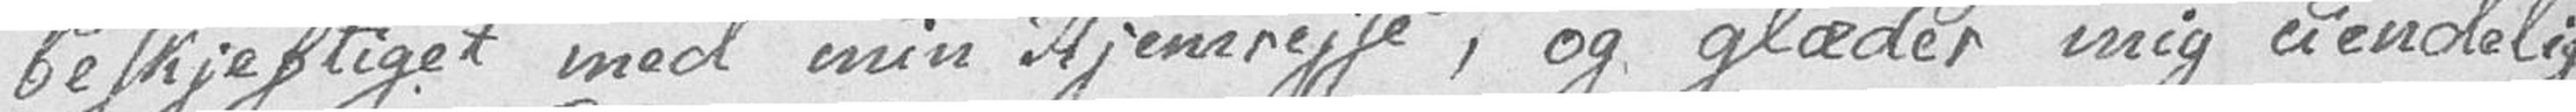beskjeftiget med min Hjemrejse, og glæder mig uendelig
